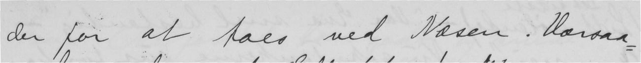der for at taes ved Næsen: Værsaa-
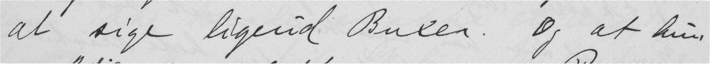at sige ligeud Buxer. Og at hun
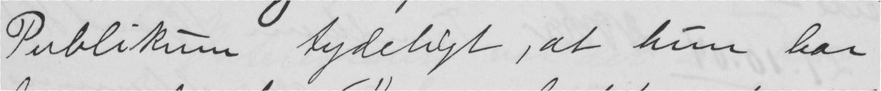Publikum tydeligt, at hun har
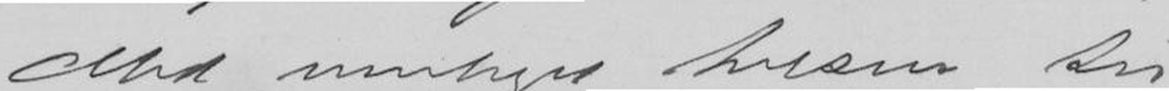Med venligt hilsen til
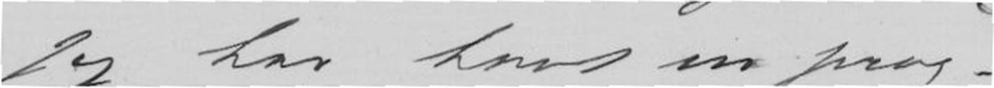Jeg har havt en præg-
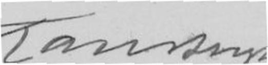Karl Bergh
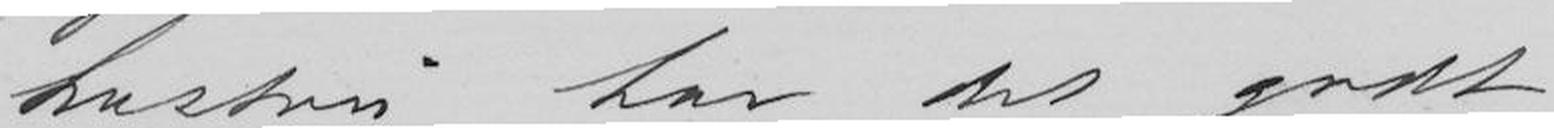hustru har det godt.
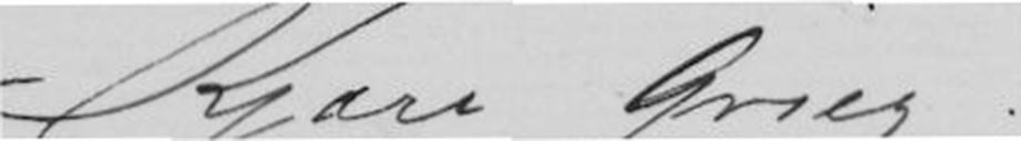Kjære Grieg!
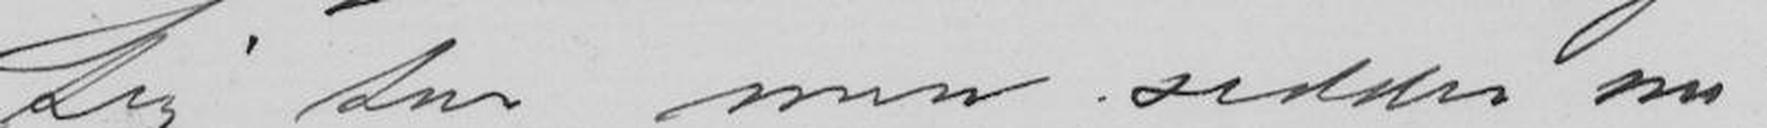tig tur men sidder nu
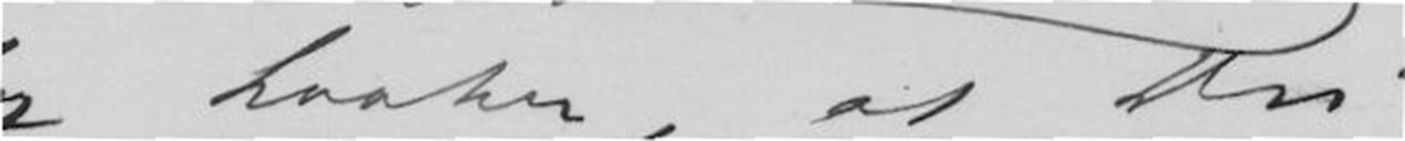Jeg haaber, at Du
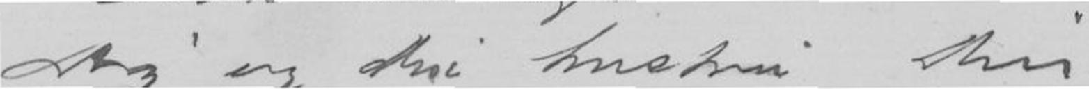Dig og Din hustru Din
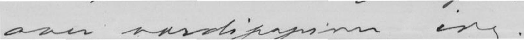over værdipapiren idag.
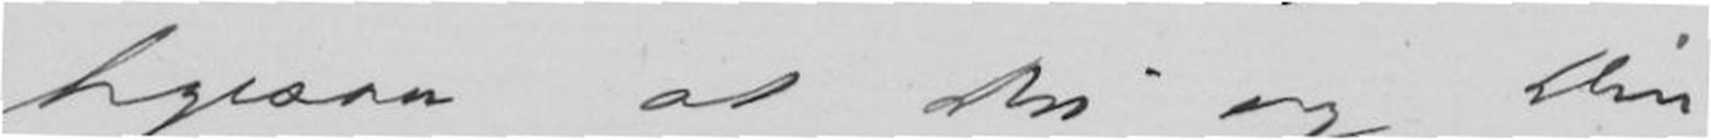ligesaa at Du og Din
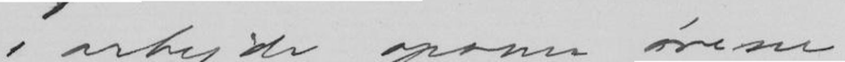i arbejde opover ørene.
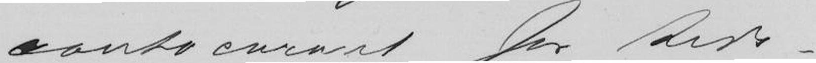contocurant for tids-
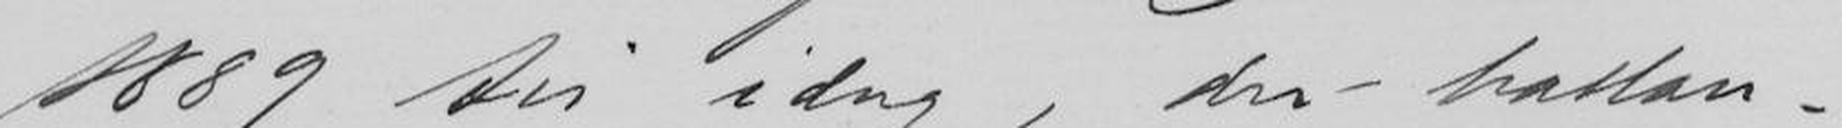1889 til idag, det ballan-
